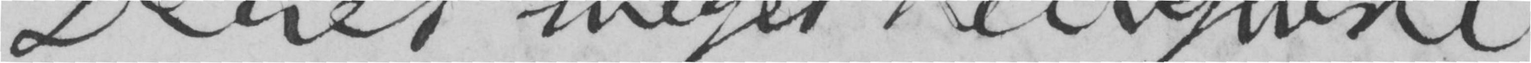Deres meget hengivne
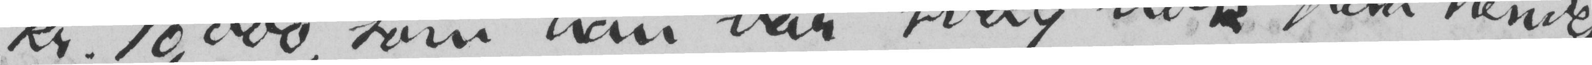kr 10,000, som han var svag nok paa hendes
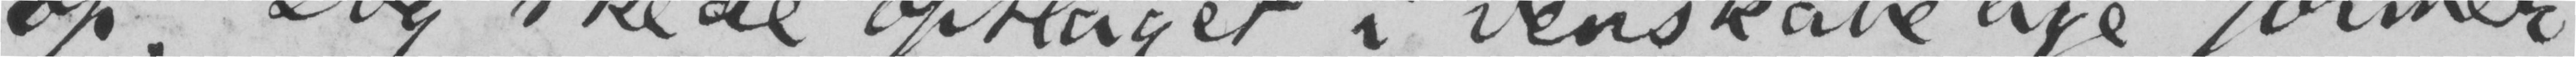op. Dog skede opslaget i venskabelige former,
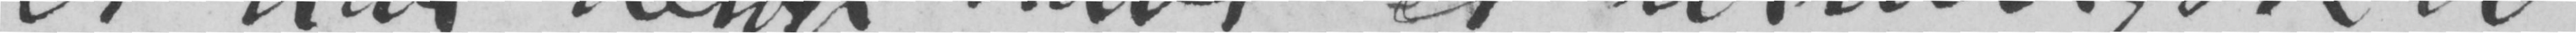Vi har netop havt et urningsselv-
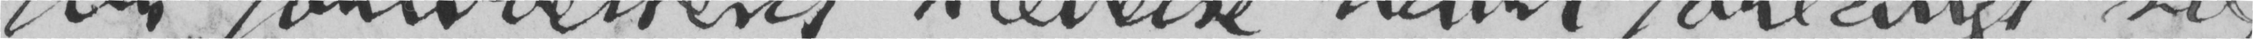for forlovelsens hævelse havde forlangt sig
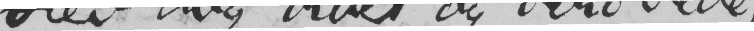blev dog troet og berøvede
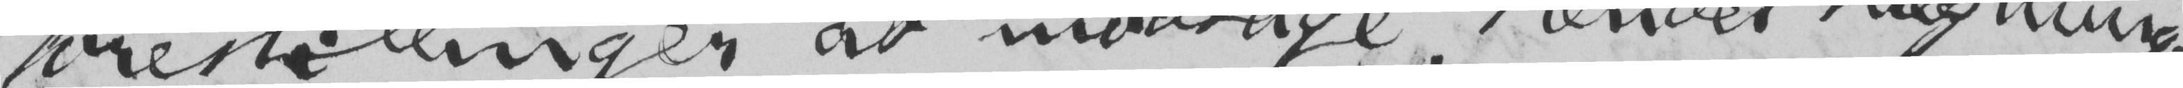forestillinger at modtage. Hendes slægtninges
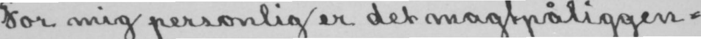For mig personlig er det magtpåliggen-
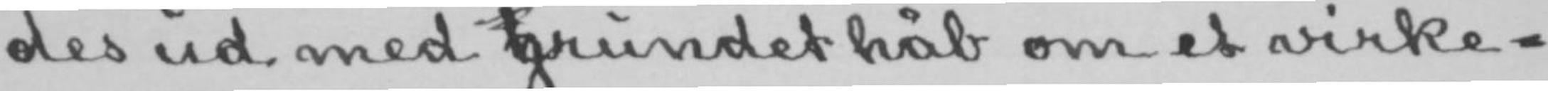des ud med grundet håb om et virke-
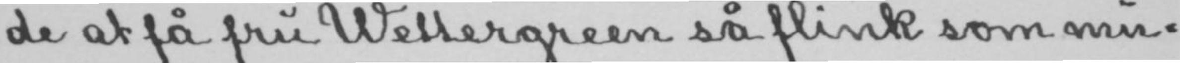de at få fru Wettergreen så flink som mu-
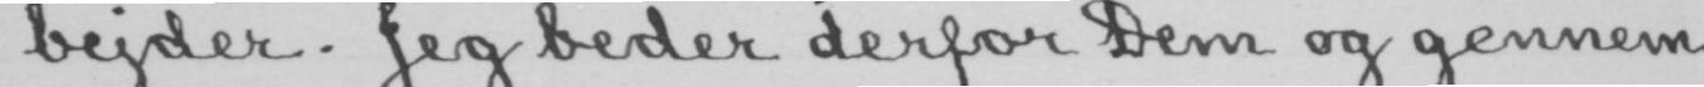bejder. Jeg beder derfor Dem og gennem
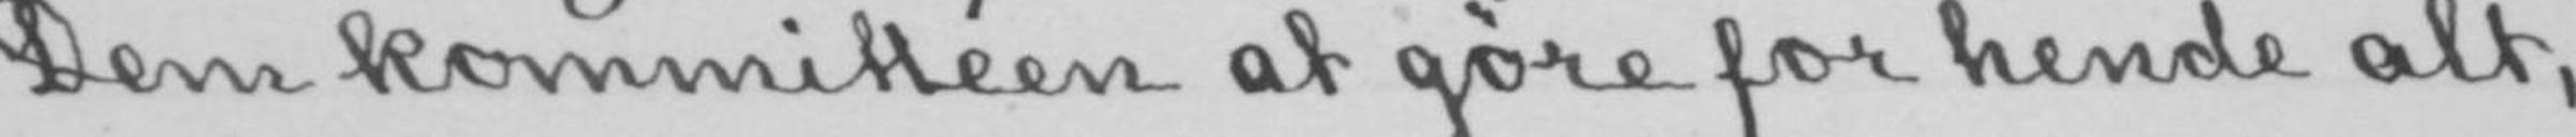Dem kommittéen at gøre for hende alt,
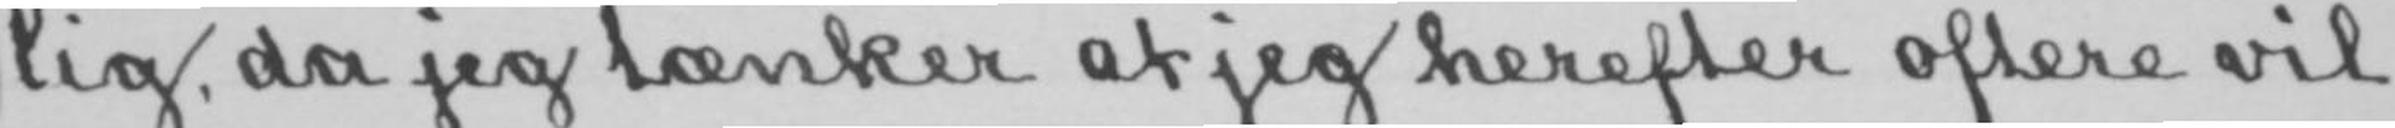lig, da jeg tænker at jeg herefter oftere vil
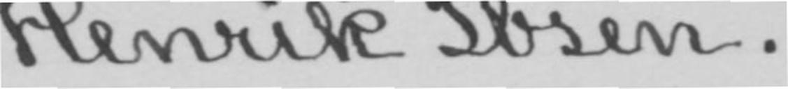Henrik Ibsen.
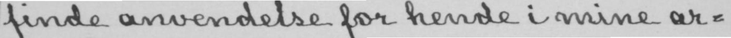finde anvendelse for hende i mine ar-
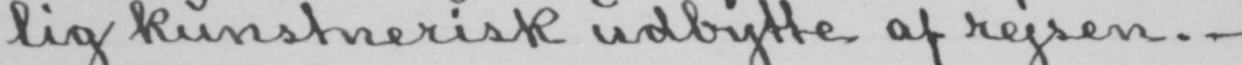lig kunstnerisk udbytte af rejsen.-
